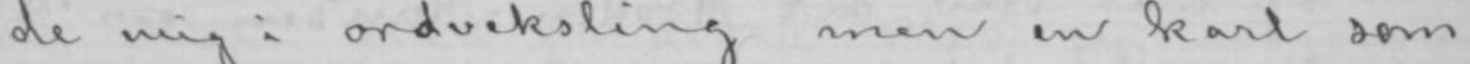de mig i ordveksling men en karl som
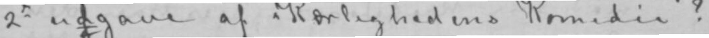2den ugdgave af «Kærlighedens Komedie»?
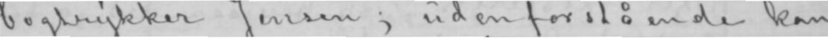bogtrykker Jensen; udenforstående kan
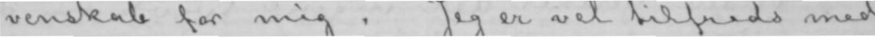venskab for mig. Jeg er vel tilfreds med
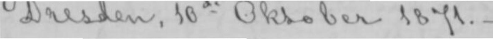Dresden, 10de Oktober 1871.-
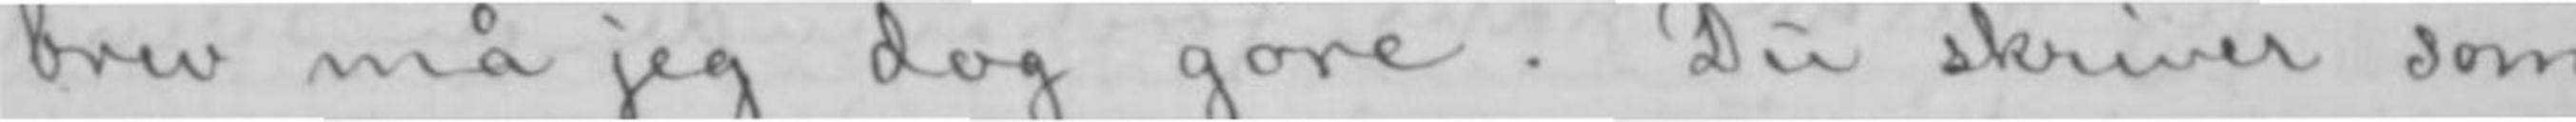brev må jeg dog gøre. Du skriver som
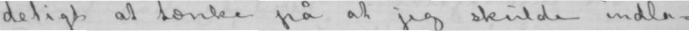deligt at tænke på at jeg skulde indla-
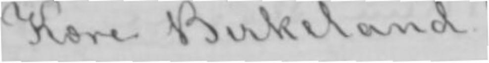Kære Birkeland!
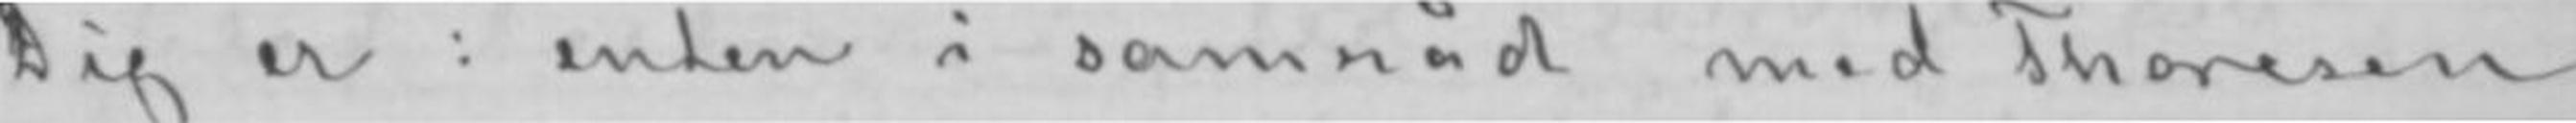Dig er: enten i samråd med Thoresen
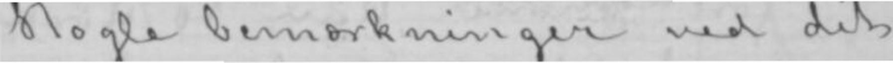Nogle bemærkninger ved dit
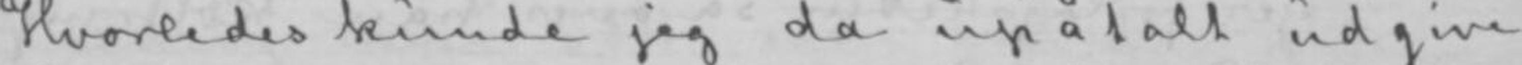Hvorledes kunde jeg da upåtalt udgive
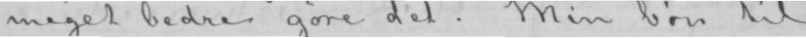meget bedre gøre det. Min bøn til
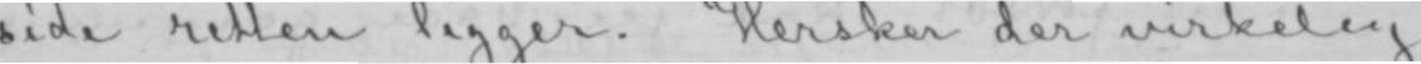side retten ligger. Hersker der virkelig
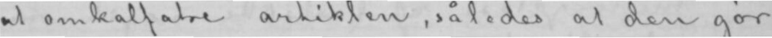at omkalfatre artiklen, således at den gør
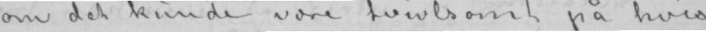om det kunde være tvivlsomt på hvis
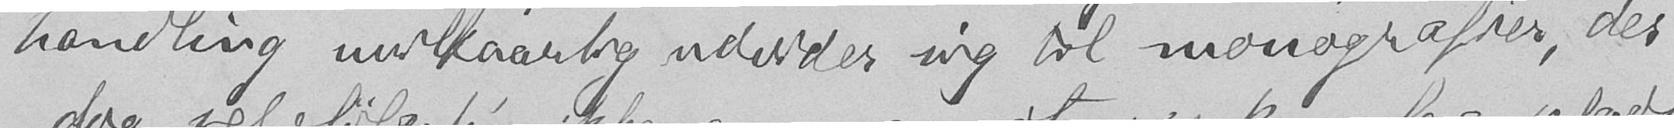handling uvilkaarlig udvider sig til monografier, der
